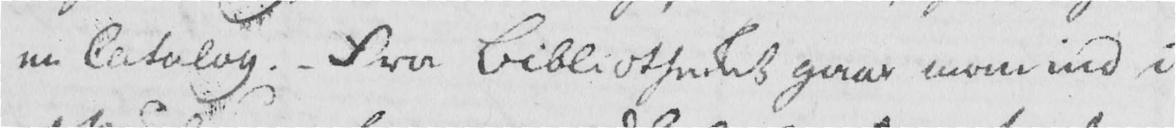en Catalog. - Fra Bibliotheket gaar man ind i
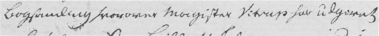Bogsamling hvorover Magister Vitrup har udgivet
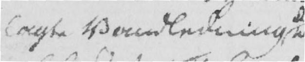lagte Vandledning der
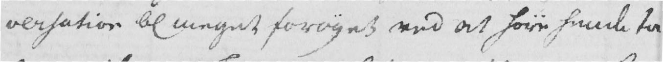versation bl meget forøget ved at høre hende ta-
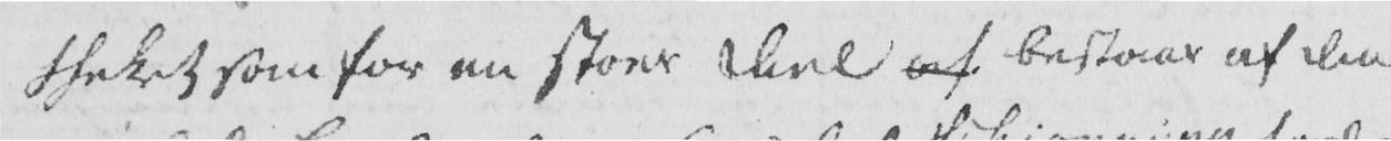theket som for en stoer Deel  bestaar af den
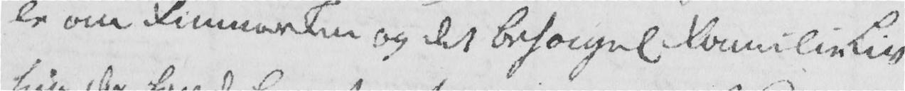le om Finmarken og det behagel Familie Liv
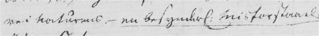ve i Naturens - en besynderl. Misforstaaelse.
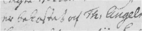er bekostet af Th. Angels
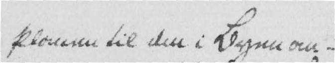Planen til den i Byen om-
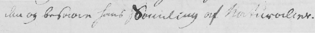den og besaae hans Samling af Naturalier
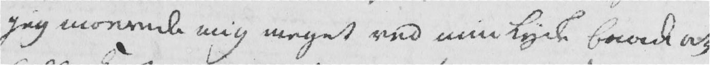jeg morede mig meget ved min Lykke baade at
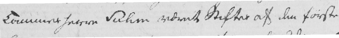Kammerherre Suhm været Stifter af den første
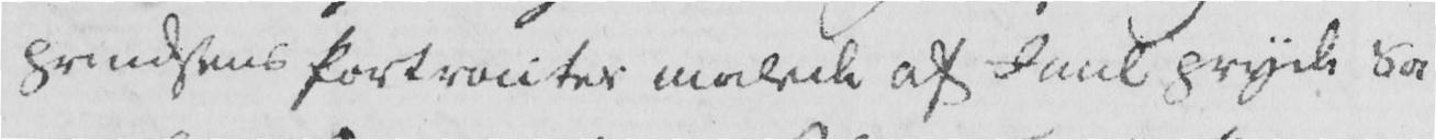prindsens portraiter malede af Juul pryde Sa-
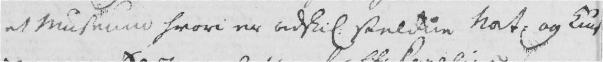et Museum hvori er adskil. sieldne Nat. og Kunst-
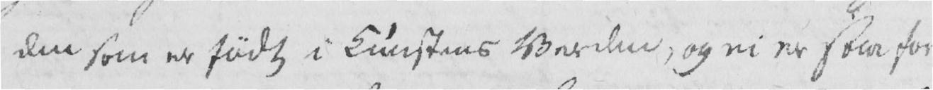den som er født i Kunstens Verden, og ei er saa for-
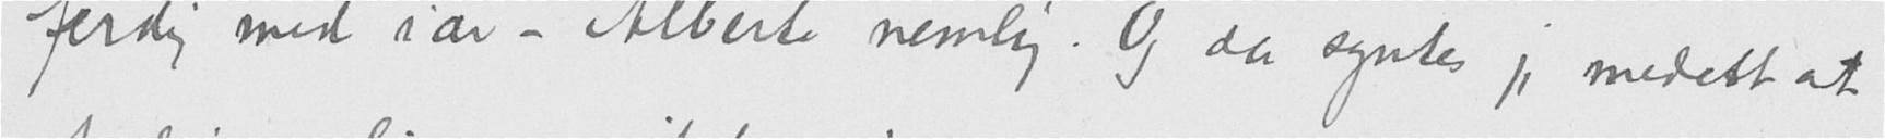ferdig med iår – Alberte nemlig. Og da syntes jeg medett at
